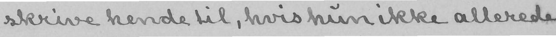skrive hende til, hvis hun ikke allerede
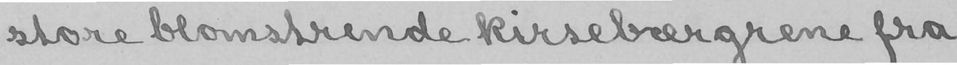store blomstrende kirsebærgrene fra
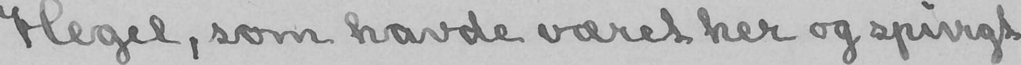Hegel, som havde været her og spurgt
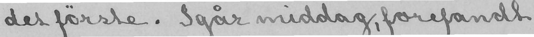det første. Igår middag, forefandt
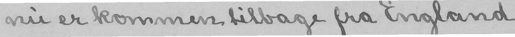nu er kommen tilbage fra England
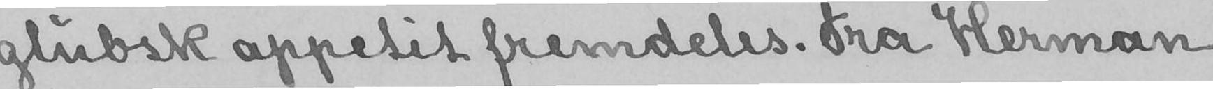glubsk appetit fremdeles. Fra Herman
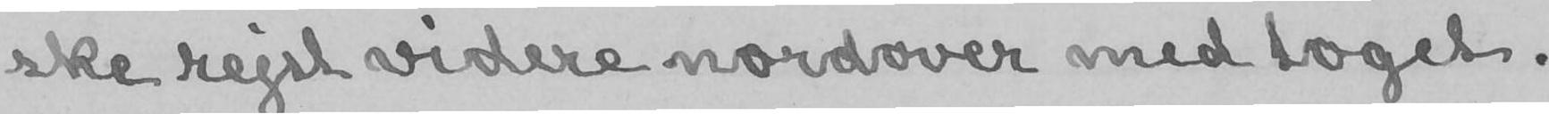ske rejst videre nordover med toget.
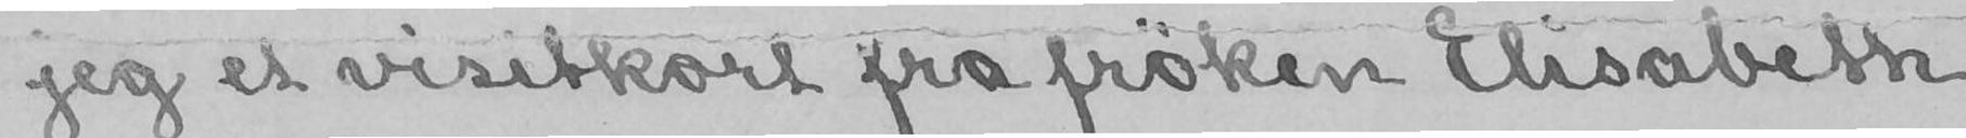jeg et visitkort fra frøken Elisabeth
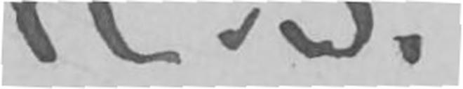H. I.
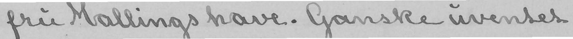fru Mallings have. Ganske uventet
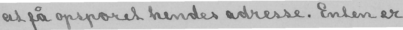at få opsporet hendes adresse. Enten er
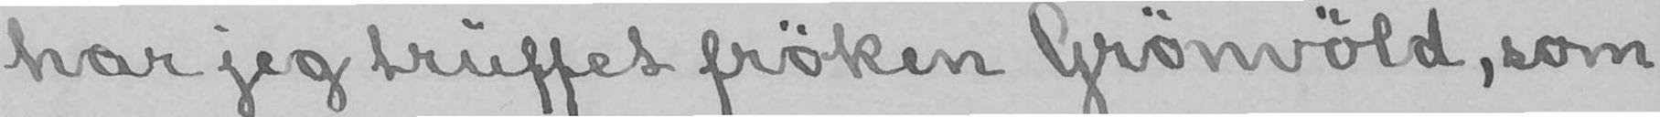har jeg truffet frøken Grønvøld, som
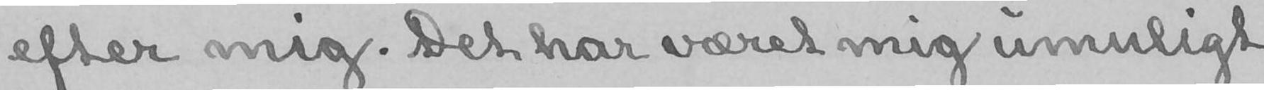efter mig. Det har været mig umuligt
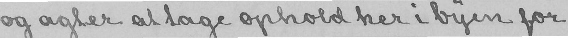og agter at tage ophold her i byen for
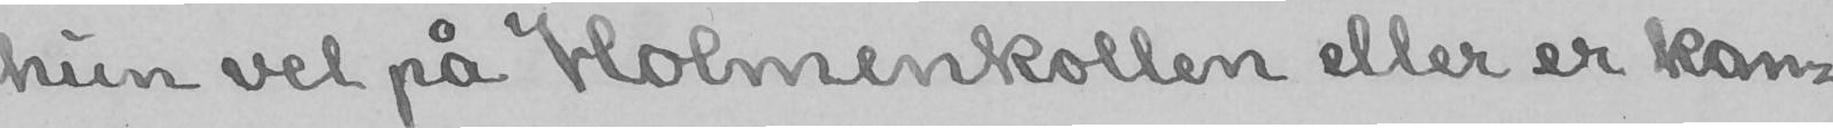hun vel på Holmenkollen eller er kan-
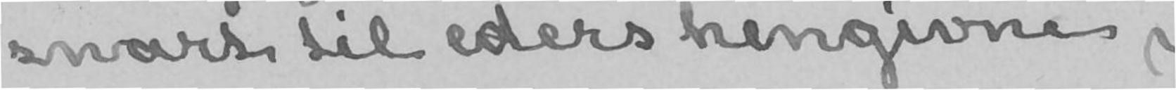snart til eders hengivne
